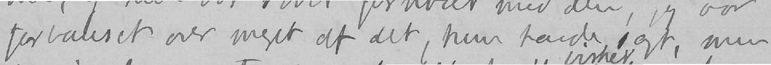forbauset over meget af det, hun havde sagt, men
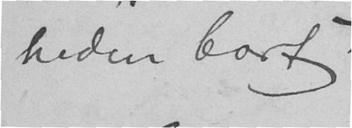heden bort]
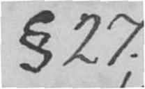§ 27.
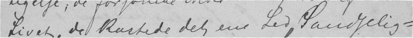Livet, de kastede det ene Led, Sandselig-
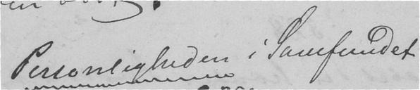Personligheden i Samfundet
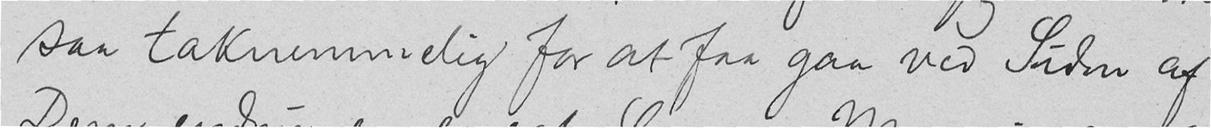saa taknemmelig for at faa gaa ved Siden af
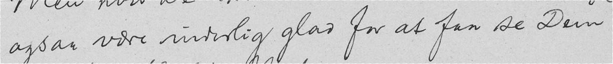ogsaa være inderlig glad for at faa se Dem
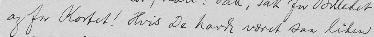og for Kortet! Hvis De havde været saa liden
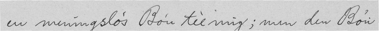en meningsløs Bøn til mig; men den Bøn
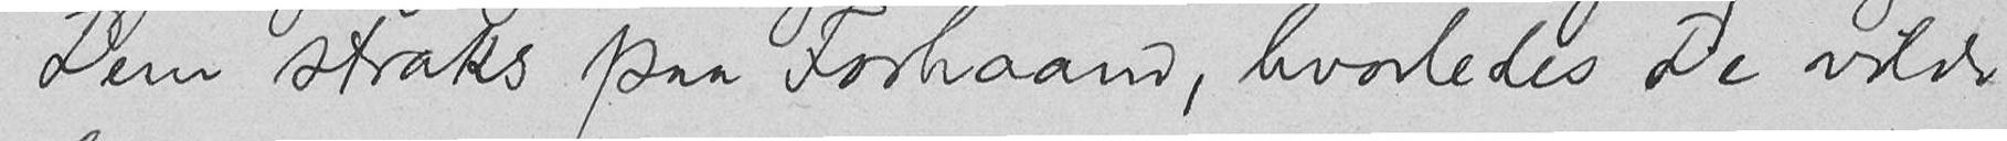Dem straks paa Forhaand, hvorledes De vilde
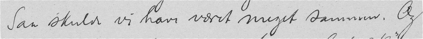Saa skulde vi have været meget sammen. Og
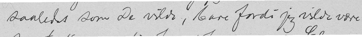saaledes som De vilde, bare fordi jeg vilde være
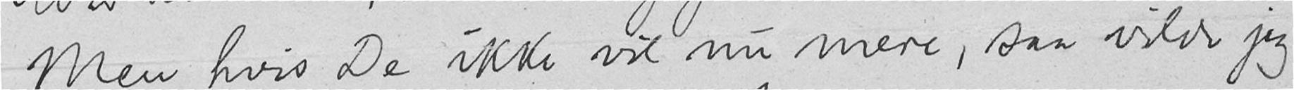Men hvis De ikke vil nu mere, saa vilde jeg
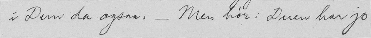i Dem da ogsaa. - Men hør: Duen har jo
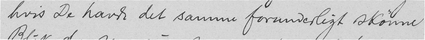hvis De havde det samme forunderligt skønne
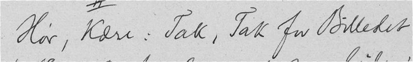Hør, Kære: Tak, Tak for Billedet
